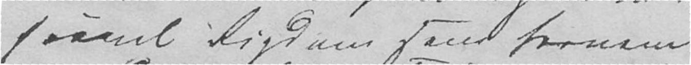saavel Rigdom som Fornem-
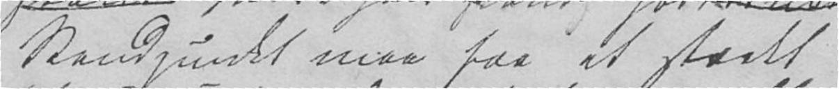Standpunkt maa faa et stærkt
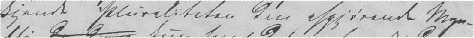kjende Pluraliteten den afgjørende Myn-
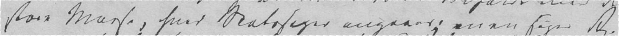store Masse, hvad Statssager angaaer; men siger A.
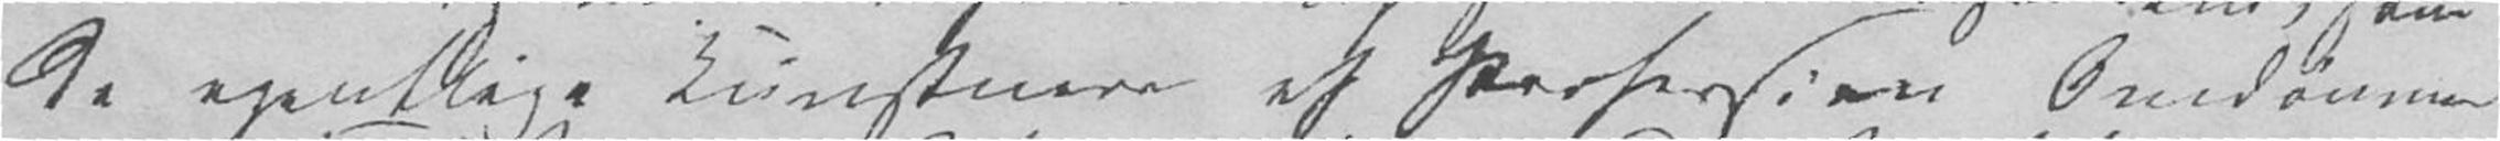de egentlige Kunstnere at Porforsien Omdømme
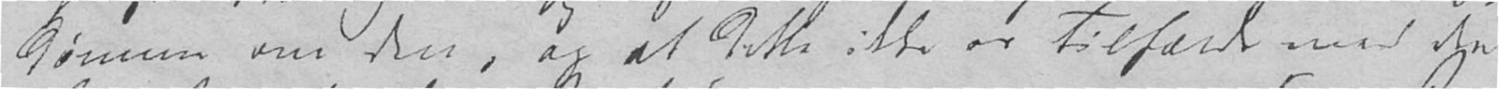dømme om den, og at dette ikke er Tilfælde med den
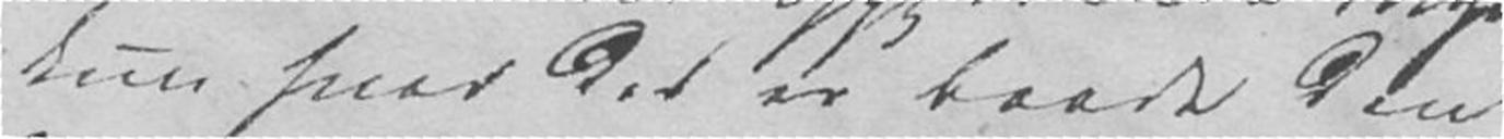kun hvad der er baade den
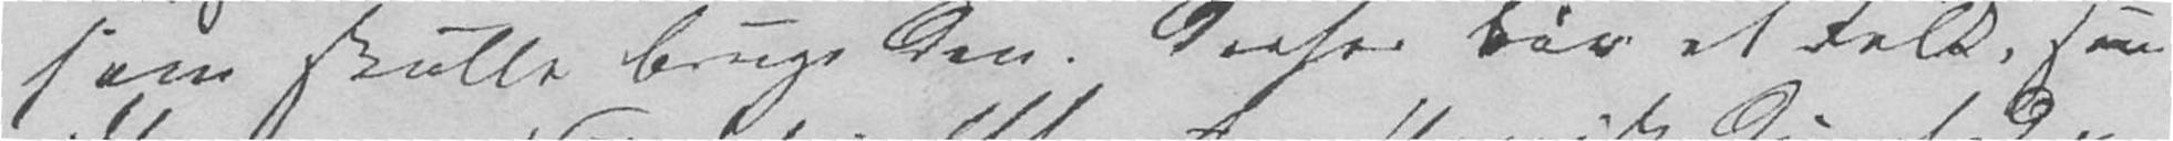som skulle bruge den. Derfor bør et Folk, som
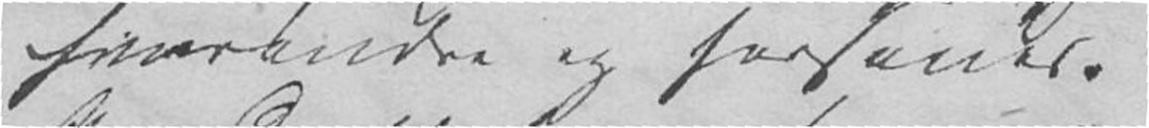hverandre og forsones.
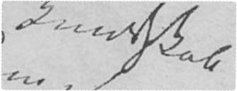Kundskab
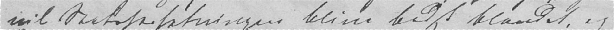vil Statsforfatningen blive bedst blandet, og
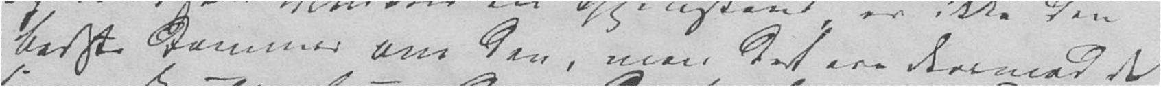bedste Dommer om den, men det ere derimod de
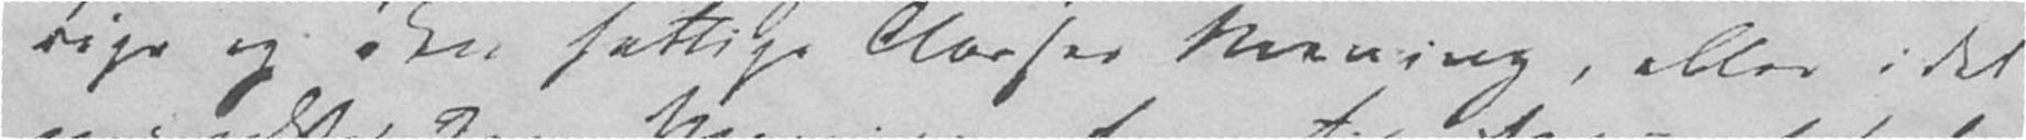rige og den fattige Classes Mening, eller i det
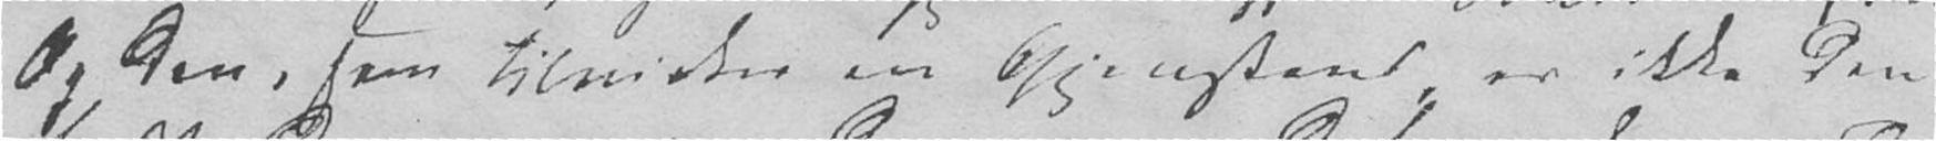Og den, som tilvirker en Gjenstand, er ikke den
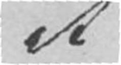at
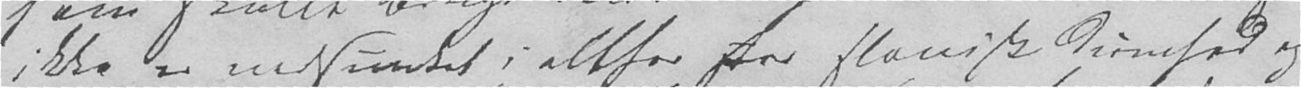ikke er nedsunket i allfor stor slavisk Dumhed og
